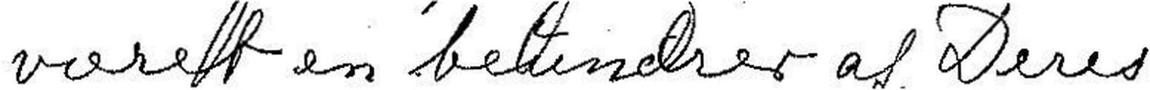været en beundrer af Deres
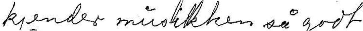kjender musikken så godt
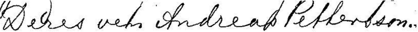Deres ven Andreas Pettersson.
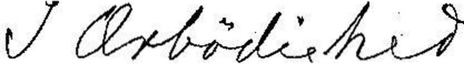I Ærbødighed
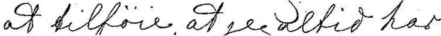at tilføie, at jeg altid har
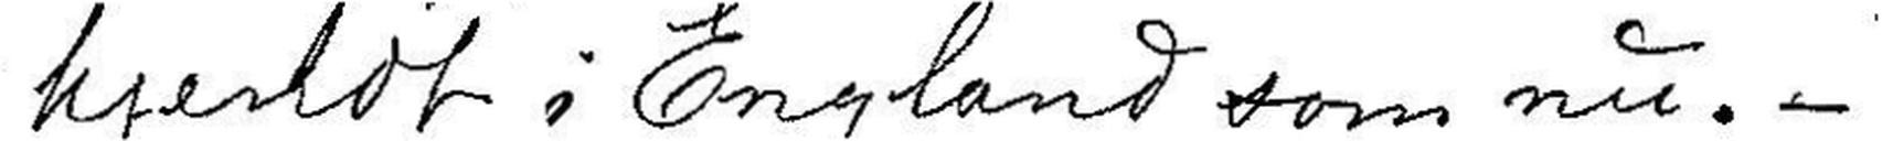kjendt i England som nu. -
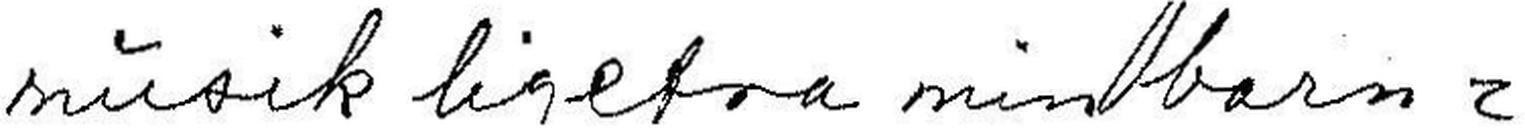musik ligefra min barn-
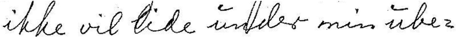ikke vil lide under min ube-
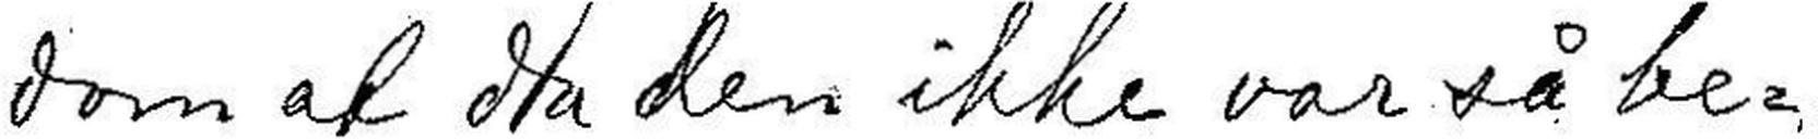dom af, da den ikke var så be-
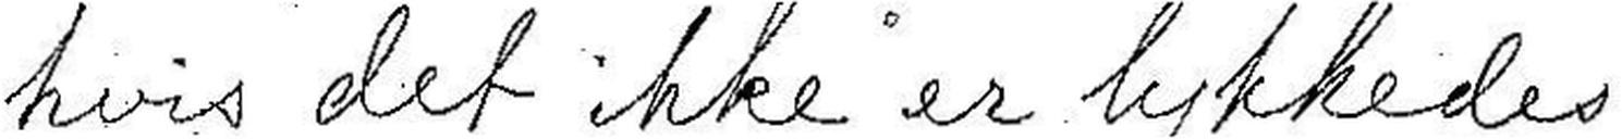hvis det ikke er lykkedes
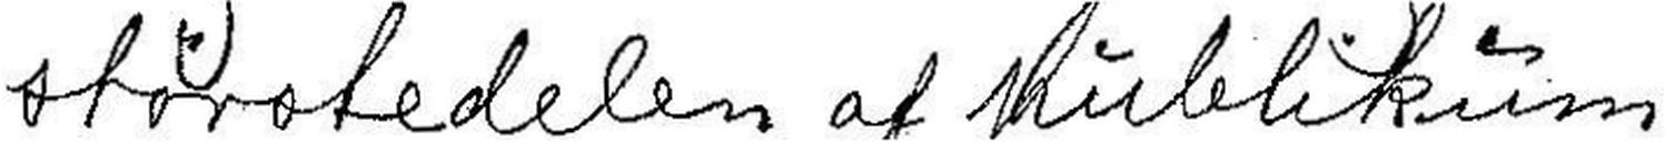størstedelen af publikum
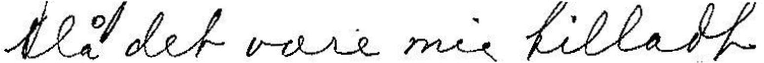Må det være mig tilladt
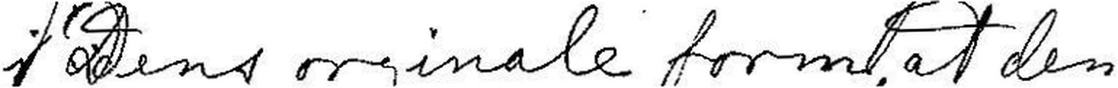i Dens orginale form, at den
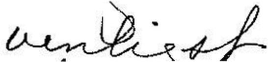venligst
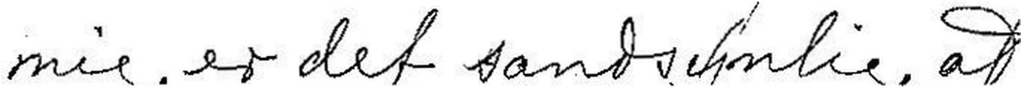mig, er det sandsynlig, at
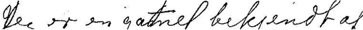Jeg er en gamel bekjendt af
